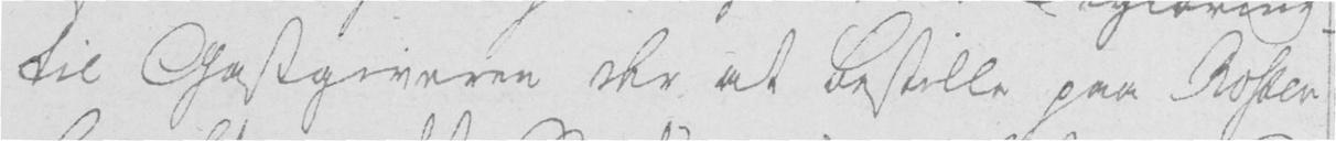til Gestgiveren der at bestille paa Rosten
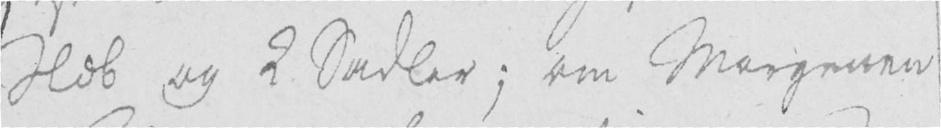Hob og 2 Sadler; om Morgenen
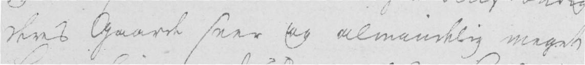deres Gaarde seer og almindelig meget
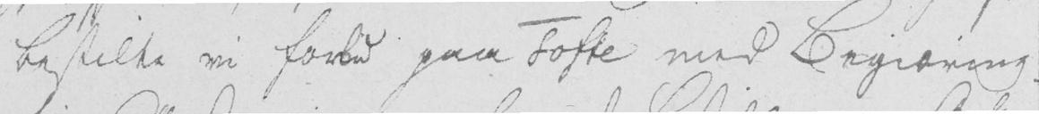bestilte vi forbe paa Tofte med Begiæring
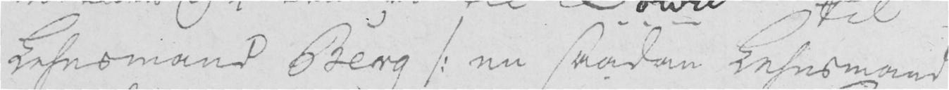Lehnsmand Berg /: en saadan Lehnsmand
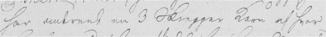har omtrent en 3 Skrepper Korn af hver
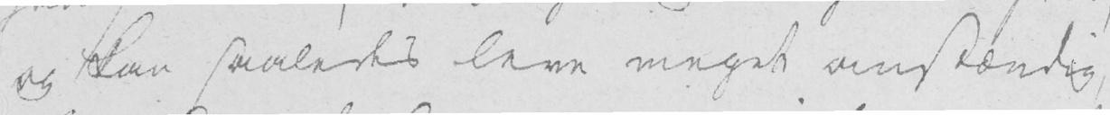og kan saaledes leve meget anstændig,
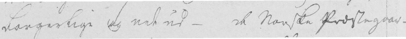borgerlige og net ud - de Norske Præstegaar-
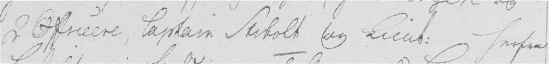2 Officeere, Captain Serbolt og Licut. Herfra
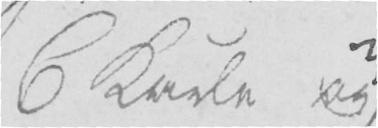6 Karle og
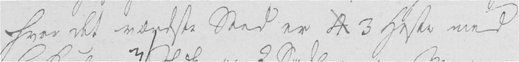hvor det væreste Sted er  3 Heste med
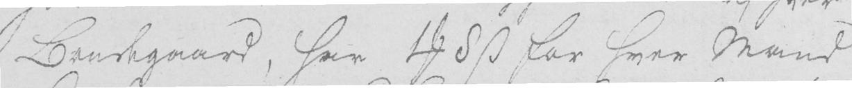Bondegaard, har 48 s for hver Mand
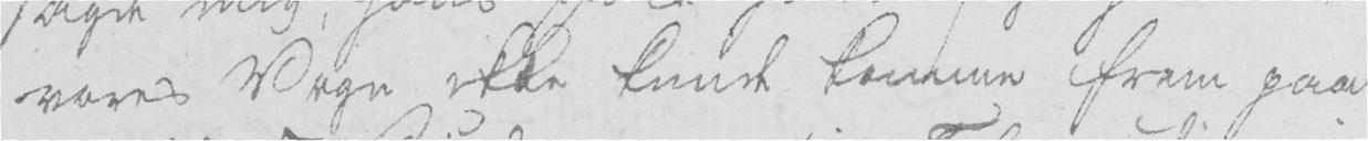vores Vogn ikke kunde komme frem paa
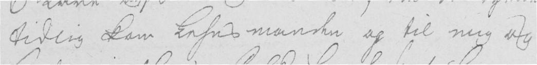tidlig kom Lehnsmanden op til mig og
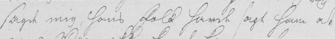sagde mig hans Folk havde sagt ham at
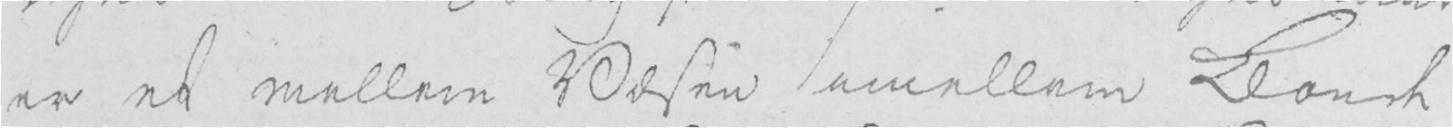er et mellem Væsen imellem Bonde
